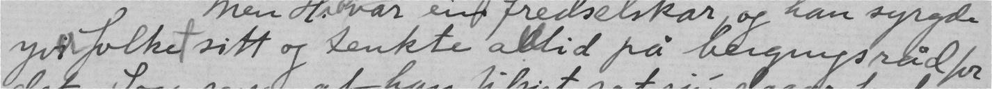yvi folket sitt og tenkte alltid på bergningsråd for
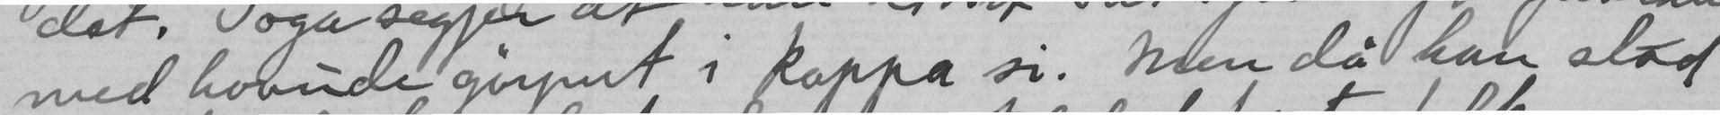med hovude gøymt i kappa si. Men då han stod
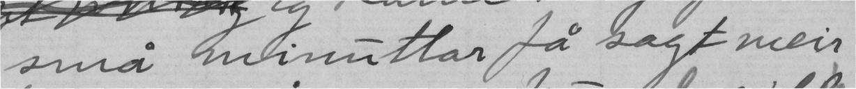små minuttar få sagt meir
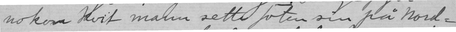nokon kvit mann sette foten sin på Nord-
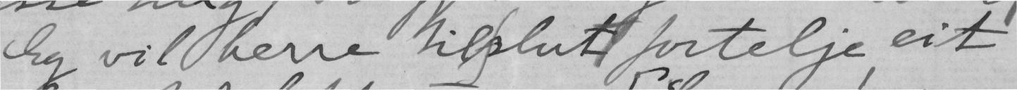Eg vil heve tilslut fortelje eit
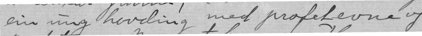ein ung hovding med profetevne og
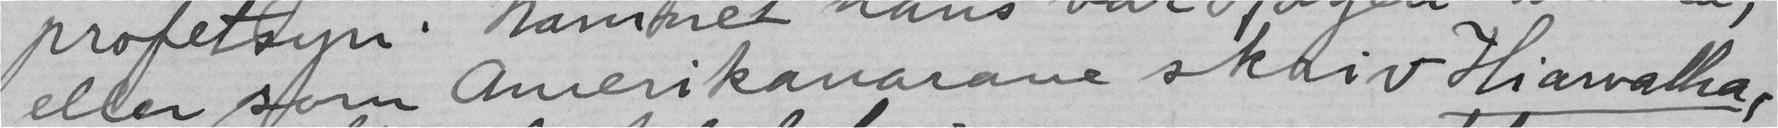eller som amerikanarane skriv Hiawatha,
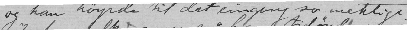og han høyrde til det eingong so mektige
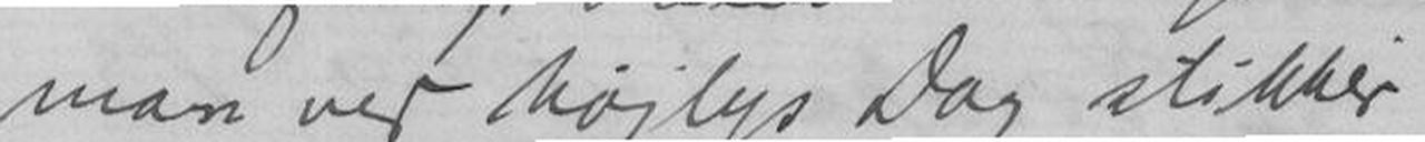man ved højlys Dag stikker
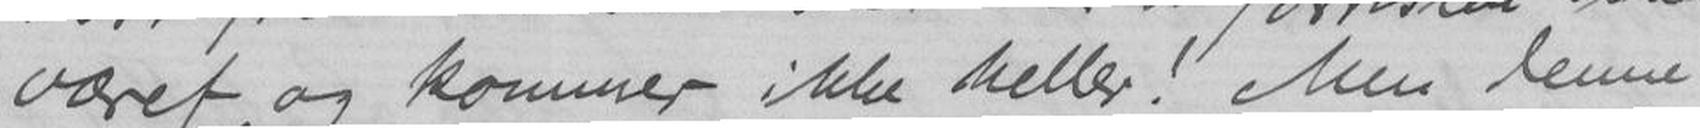været og kommer ikke heller! Men denne
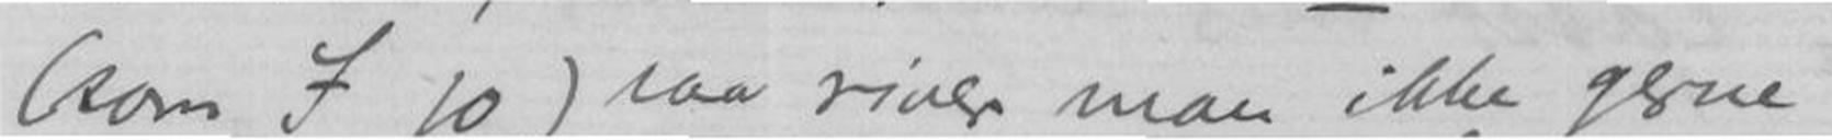(som I jo) saa river mam ikke gerne
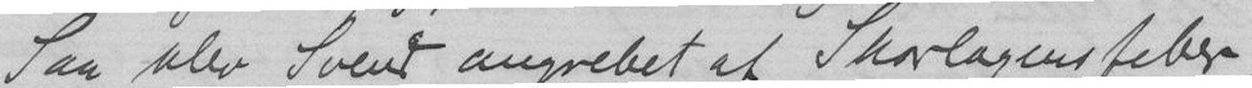Saa blev Svend angrebet af Skalagensfeber
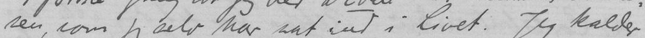sen, som jeg selv har sat ind i Livet. Jeg kalder
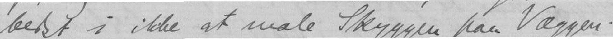bedst i ikke at male Skyggen paa Væggen.
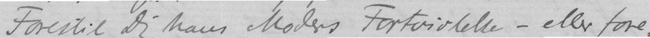Forestil Dig hans Moders Fortvivlelse - eller fore-
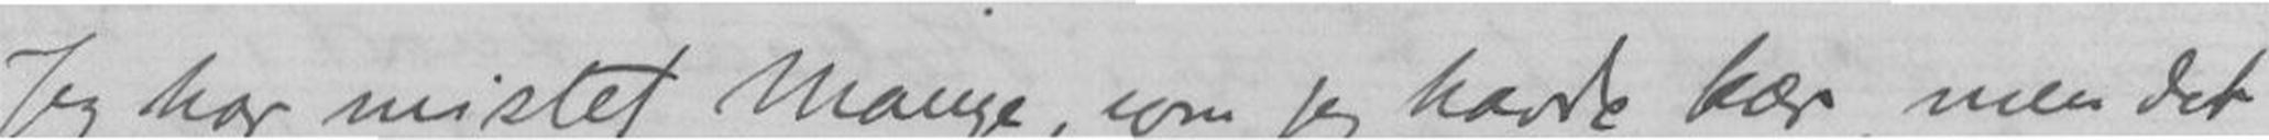Jeg har mistet Mange, som jeg havde kær, men det
